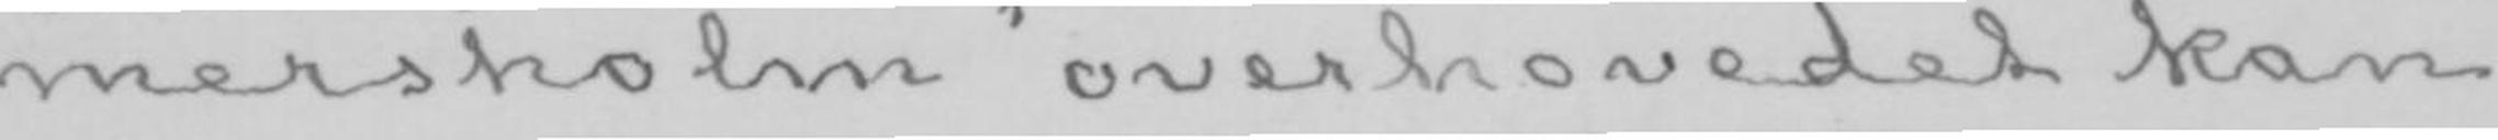mersholm» overhovedet kan
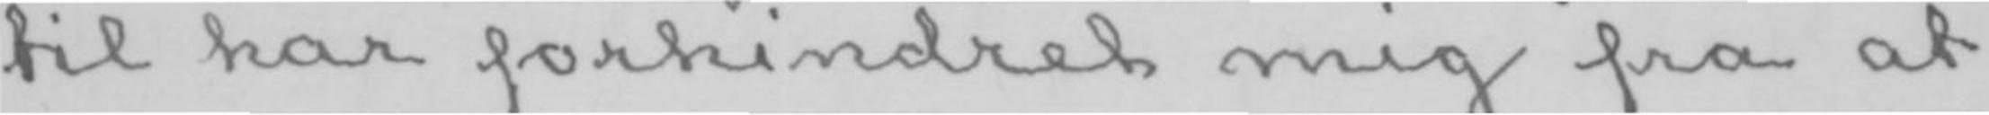til har forhindret mig fra at
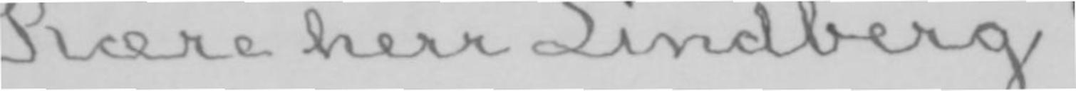Kære herr Lindberg!
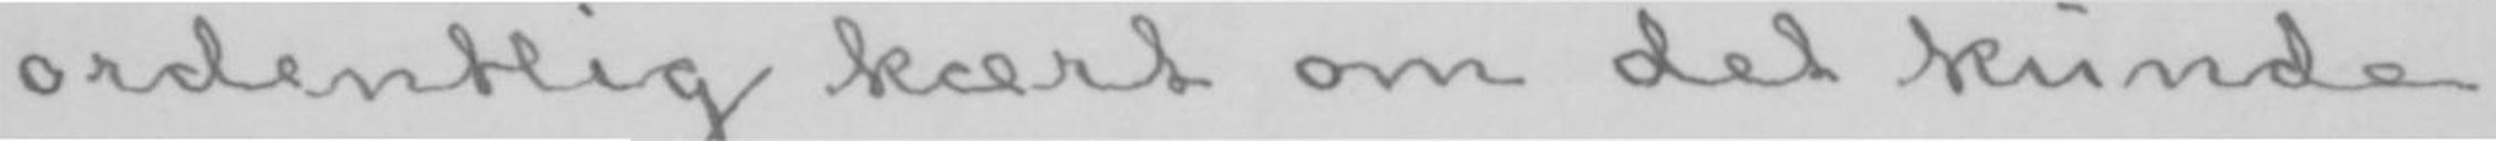ordentlig kært om det kunde
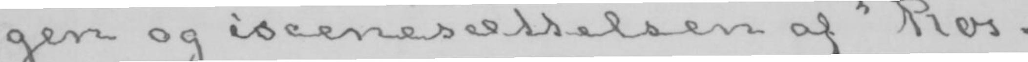gen og iscenesættelsen af «Ros-
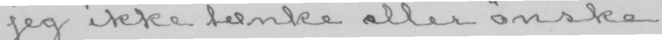jeg ikke tænke eller ønske
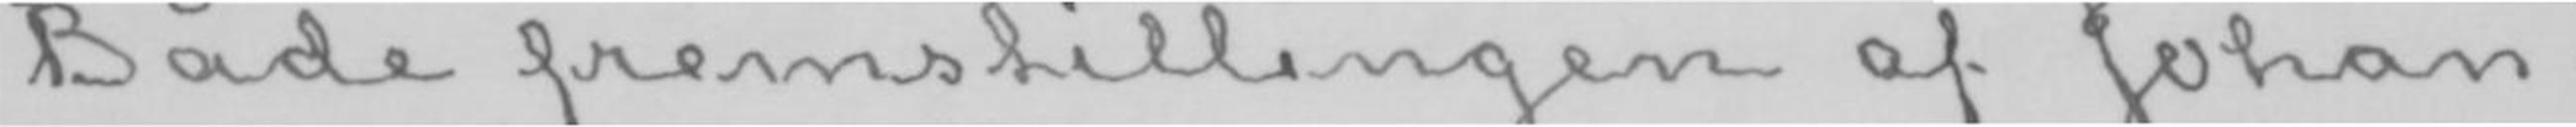Både fremstillingen af Johan-
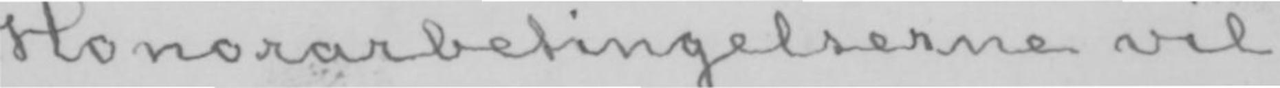Honorarbetingelserne vil
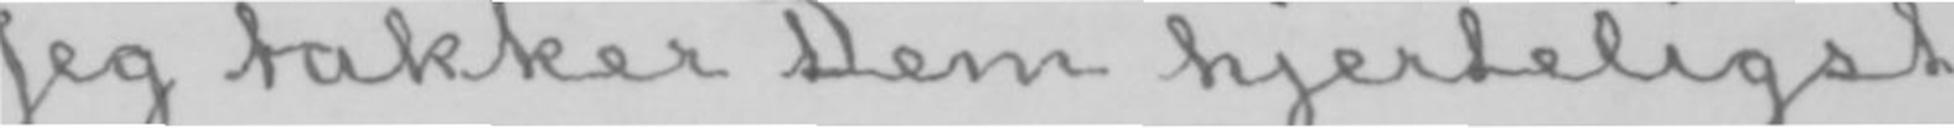Jeg takker Dem hjerteligst
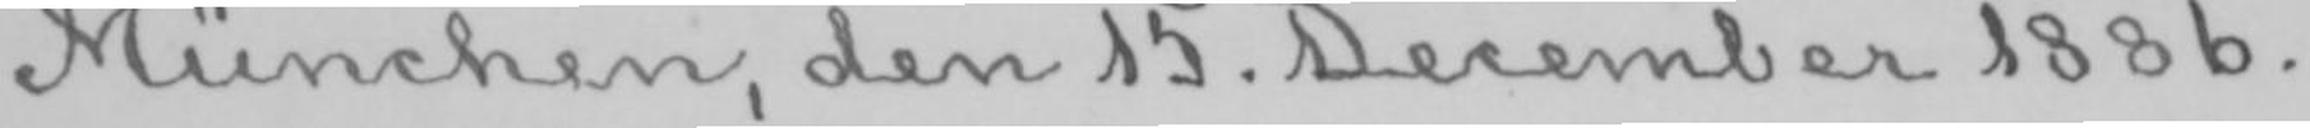München, den 15. December 1886.
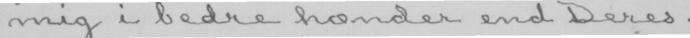mig i bedre hænder end Deres.
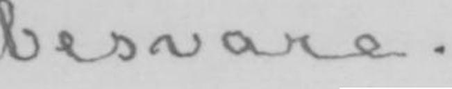besvare.
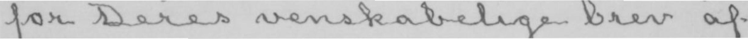for Deres venskabelige brev af
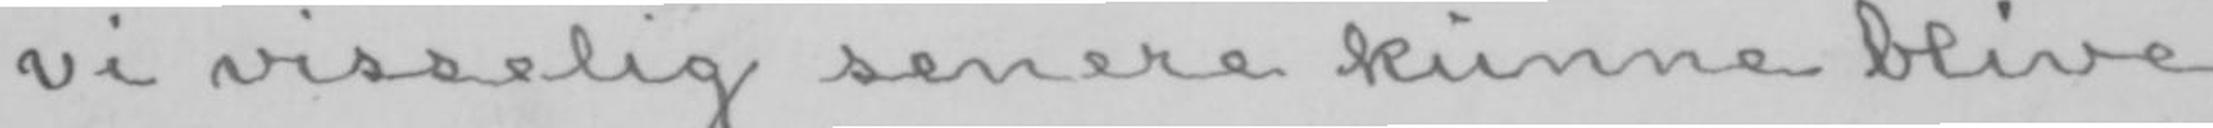vi visselig senere kunne blive
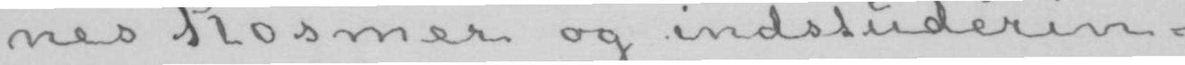nes Rosmer og indstuderin-
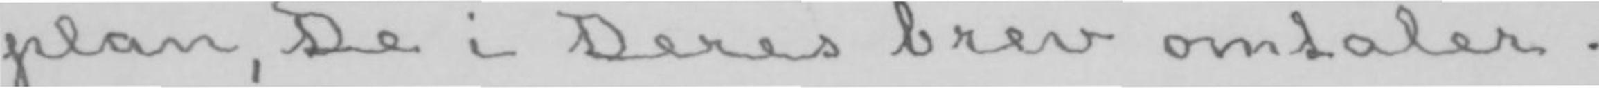plan, De i Deres brev omtaler.
